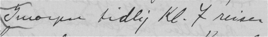Imorgen tidlig Kl. 7 reiser
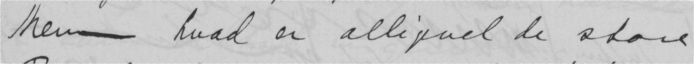Men- hvad er alligevel de store
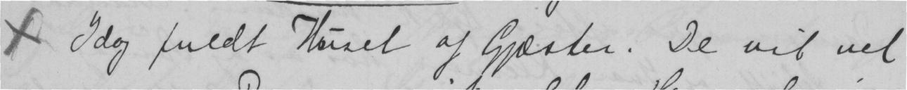x Idag fuldt Huset af Gjæster. De vil vel
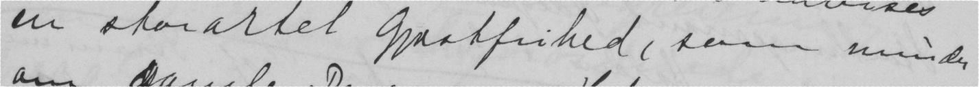en storartet gjæstfrihed, som minder
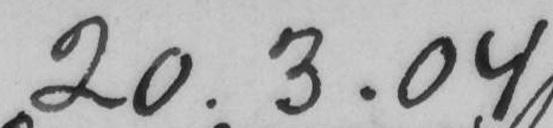20.3.04
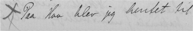x Paa Haa blev jeg hentet til
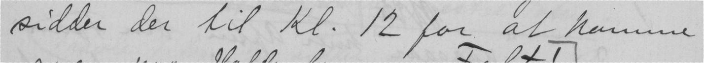sidder der til Kl. 12 for at komme
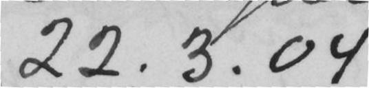22.3.04
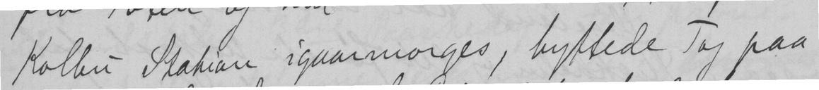Kolbu Station igaarmorges, byttede Tog paa
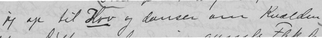jeg op til Hov og danser om Kvælden
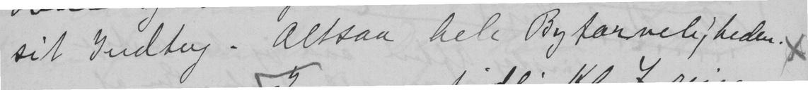sit Indtog. Altsaa hele Bytarveligheden. x
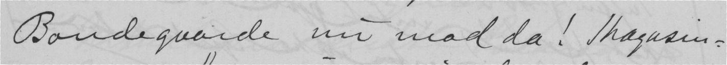Bondegaarde nu mod da! Magasin-
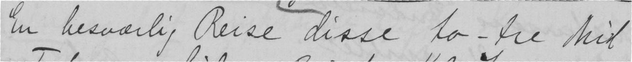En besværlig Reise disse to -tre mil
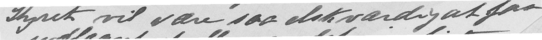Styret vil være saa elskværdig at faa
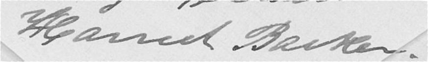Harriet Backer.
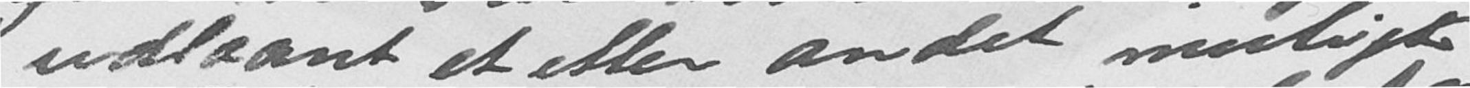udlaant et eller andet muligt
